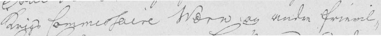Krigs Commissaire Wærn og andre frievil-
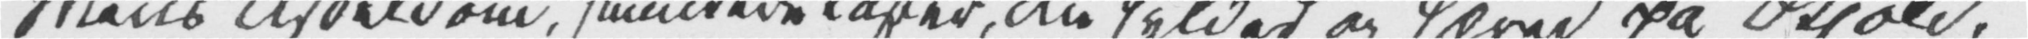Mens Usseldom, sminkede Laster, du hylded og hæved på Skjold.
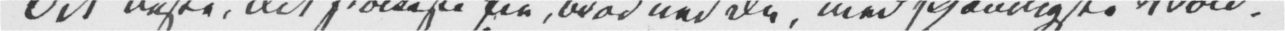Dit beste, dit stolteste Eie, brød ned du, med skjændigste Vold.
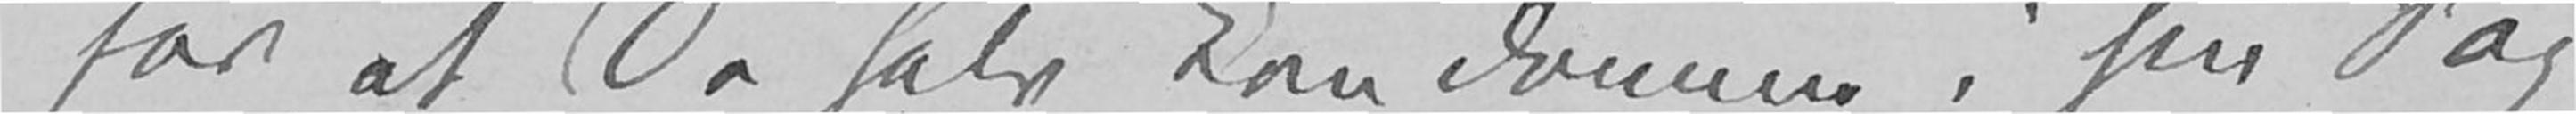for at De selv kan dømme i hin Sag[.]
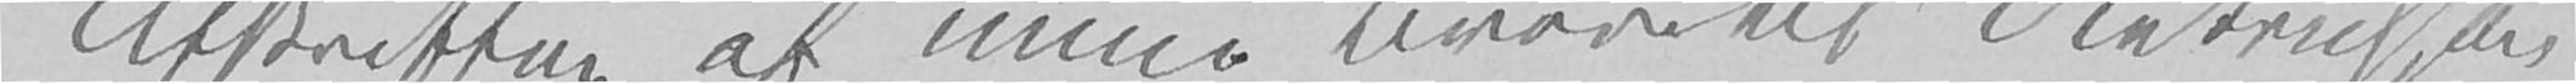Afskriften af mine Breve til Dietrichson
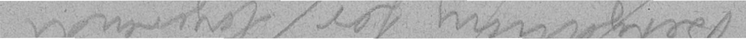Betydning for logerende
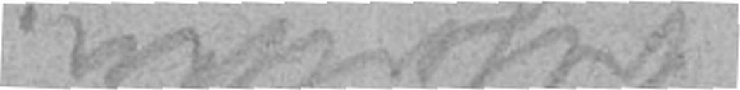befolkn.
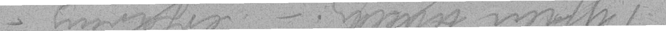Typen uheldig? erfaring -
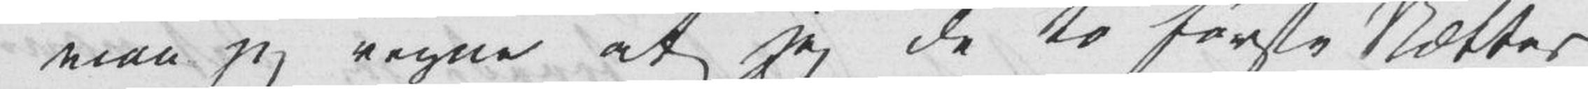maa jeg regne at jeg de to første Nætter
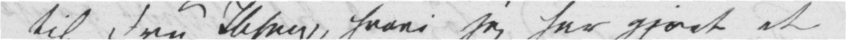til Fru Ibsen, hvori jeg har gjort et
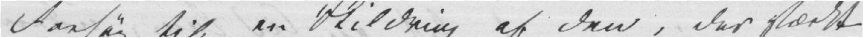Forsøg til en Skildring af den, der stærkt
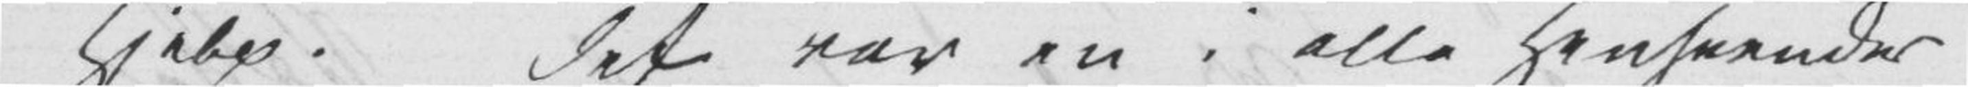Hjelp. Det var en i alle Henseender
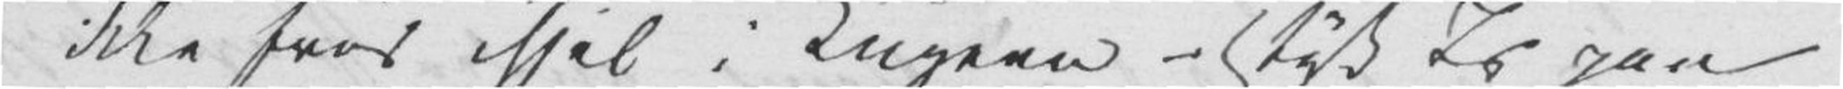ikke frøs i hjel i Kupeen – (tyk Is paa
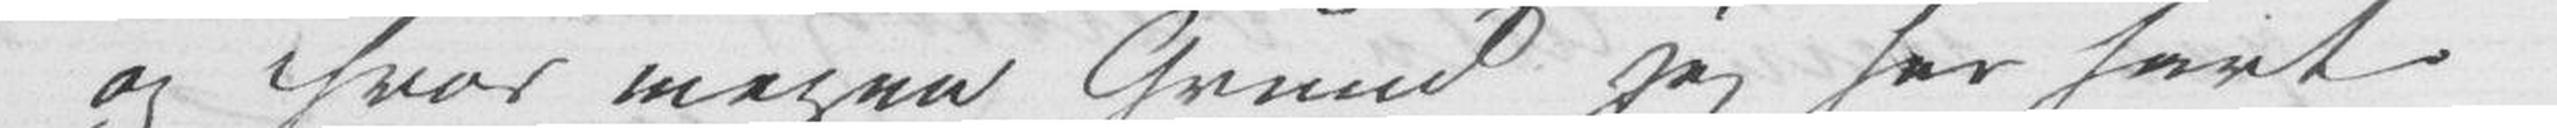og hvor megen Grund jeg har havt
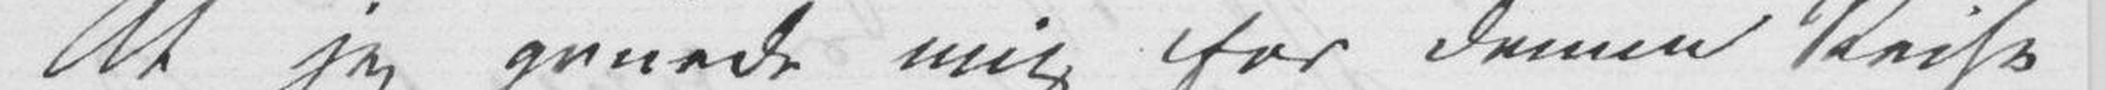At jeg gruede mig for denne Reise
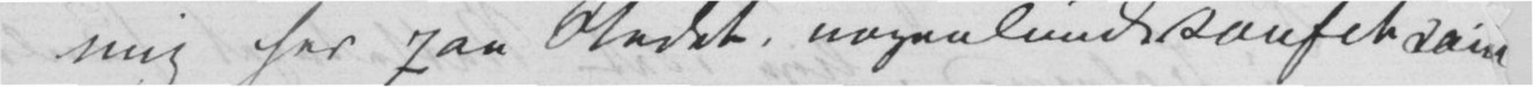mig her paa Stedet, nogenlunde sauf et sain[.]
